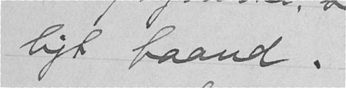ligt baand.
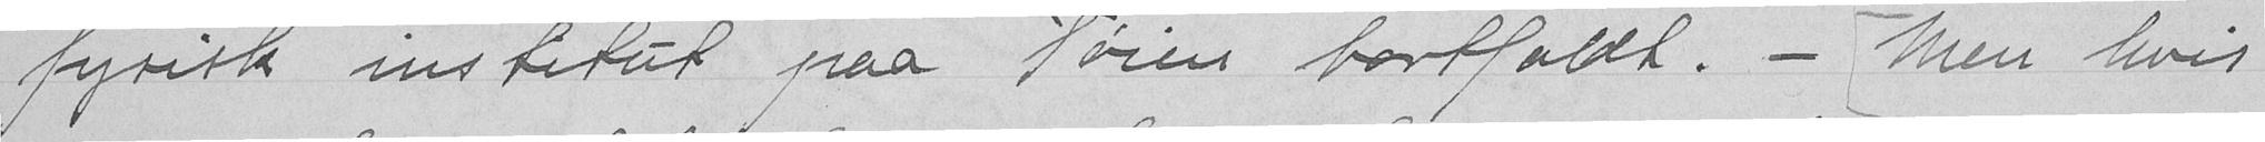fysisk institut paa Tøien bortfaldt. - Men hvis
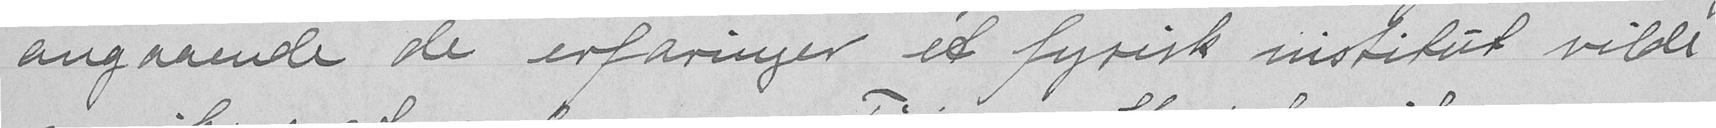angaaende de erfaringer et fyrisk institut vilde
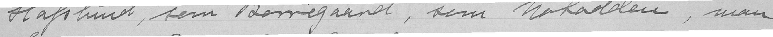Hafslund, som Borregaard, som Notodden, man
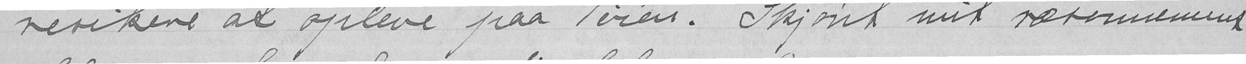risikere at opleve paa Tøien. Skjønt mit ræsonnement
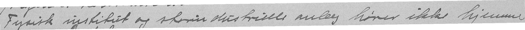Fysisk institut og stor industrielle anlæg hører ikke hjemme
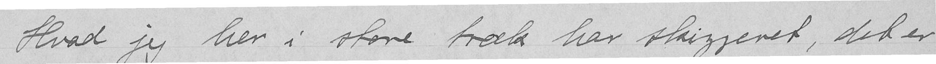Hvad jeg her i store træk har skizzeret, det er
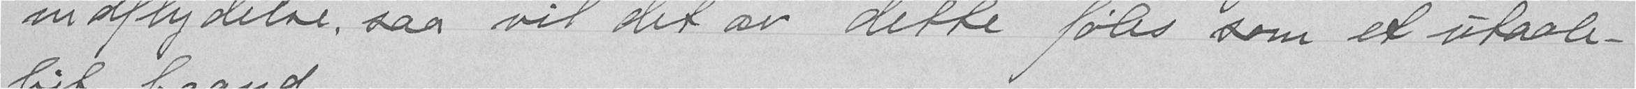indflydelse, saa vil det av dette føles som et utaale-
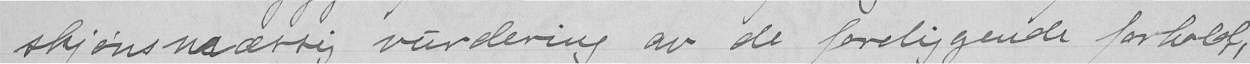skjønsmæssig vurdering av de foreliggende forhold,
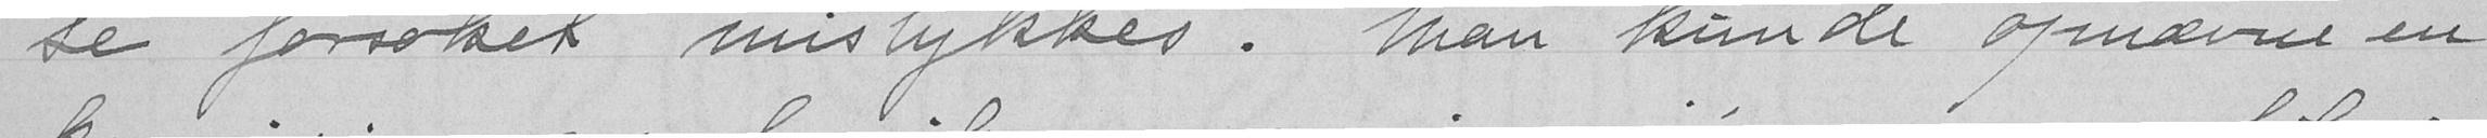se forsøket nislykkes. Man kunde opævne en
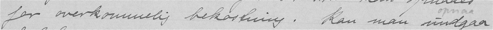for overkommelig bekostning. Kan man undgaa
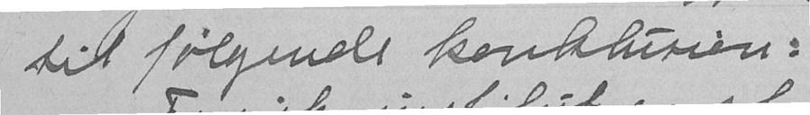til følgende konklusion:
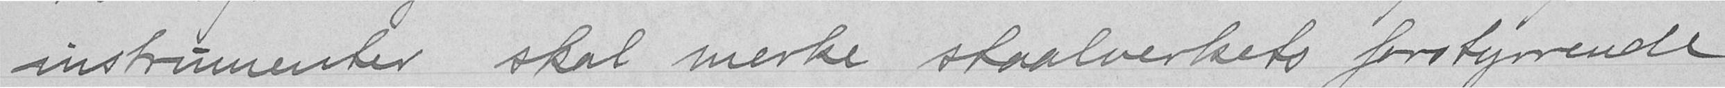instrumenter skal merke staalverkets forstyrrende
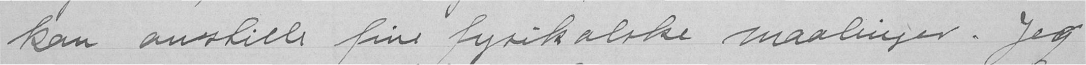kan ansstille sine fysikalske maalinger. Jeg
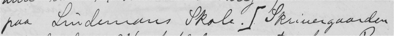paa Lindemans Skole. Skrivergaarden
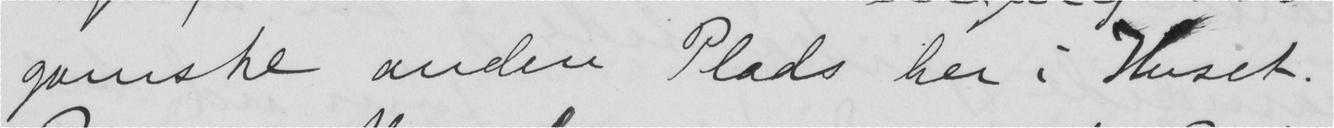ganske anden Plads her i Huset.
